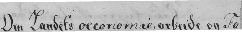Om Landets oeconomie, arbeide og Fa-
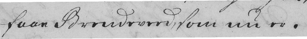faae Brendeveed, som nu er.
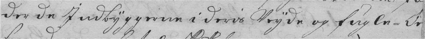der de Indbyggerne i deris Veyde og fugle-
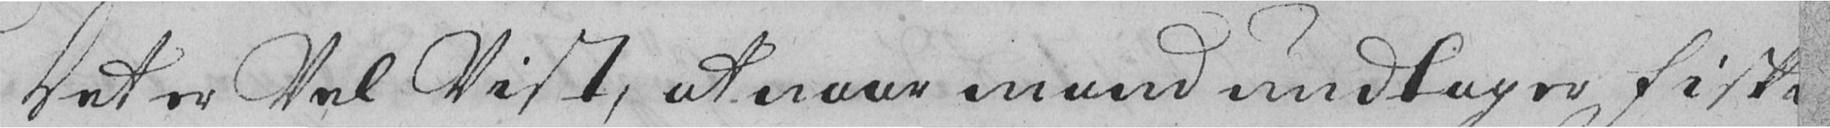Det er Vel Vist, at naar mand undtager Fiske-
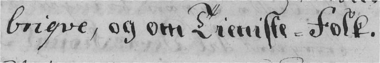briqve, og om Tieniste-folk.
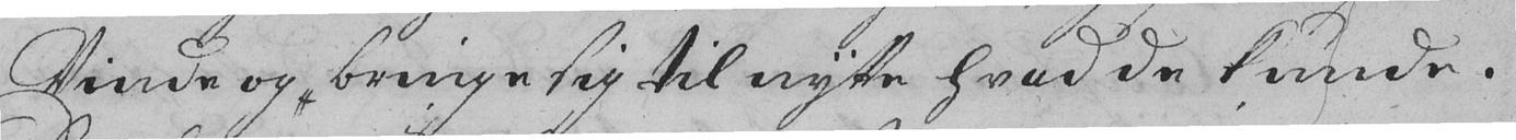Vinde og bringe sig til nytte hvad de kunde.
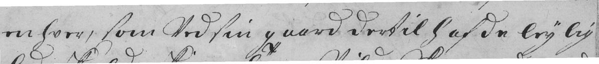en hver, som Ved sin gaard dertil hafde leylig-
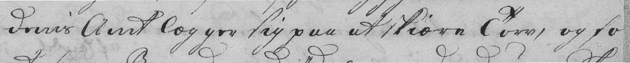denis Amt lægger sig paa at skiære Torv, og for
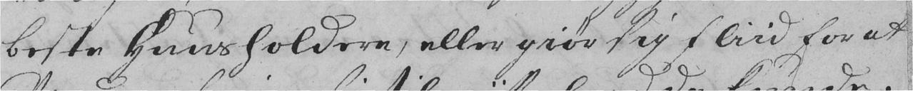beste Huusholdere, eller giør sig fliid for at
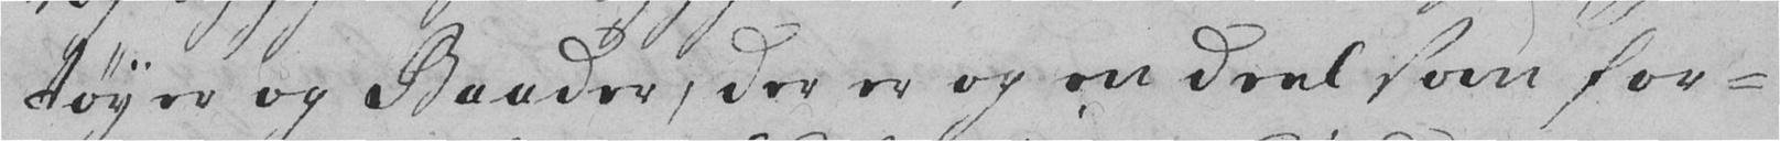tøyer og Baader, der er og en deel som for-
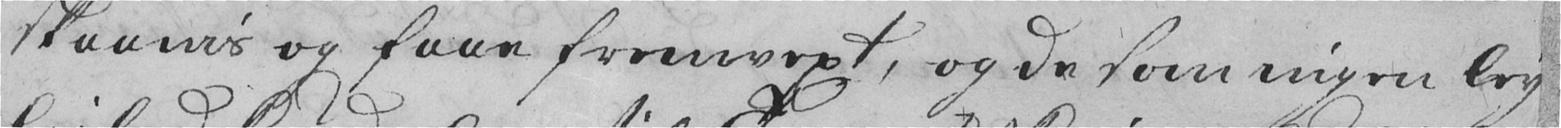skaanis og faae fremvext, og de som ingen ley-
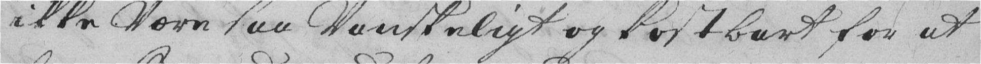ikke være saa Vanskeligt og kostbart for at
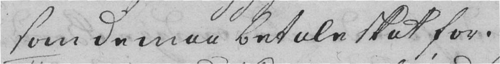som de maa betale skat for.
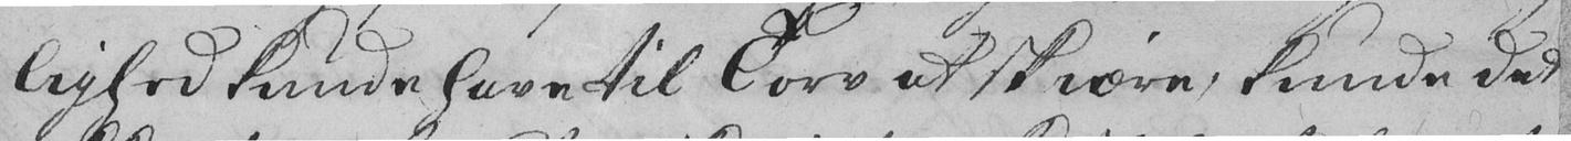lighed kunde have til Torv at skiære, kunde det
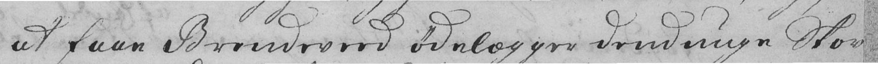at faae Brendeveed ødelægger dend unge Skov
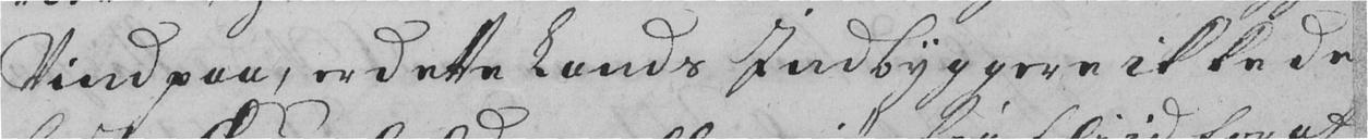Vind paa, er dette Lands Indbyggere ikke de
